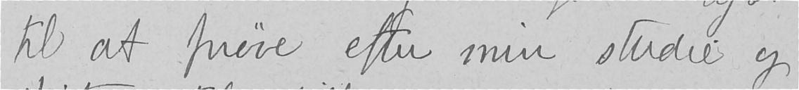til at prøve efter min studie og
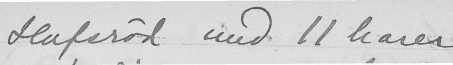Hafsrød med 11 harer
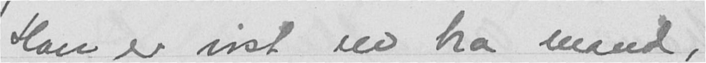Han er vist en bra mand,
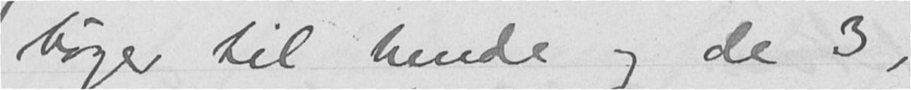bøger til hende og de 3,
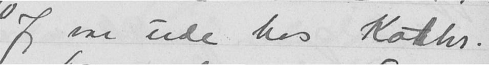Jeg var ude hos Kathr.
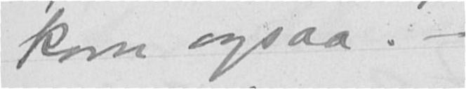kom ogsaa. -
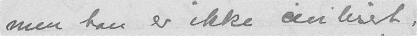men han er ikke civilisert,
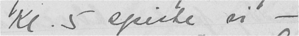Kl. 5 spiste vi -
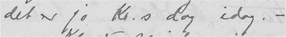det er jo Kr.s dag idag. -
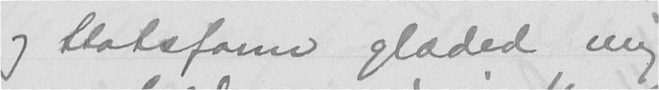og Statsform glæded mig
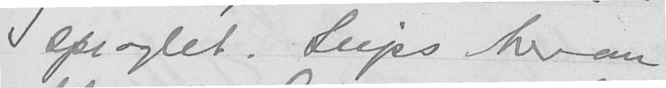spraglet. Seips her om
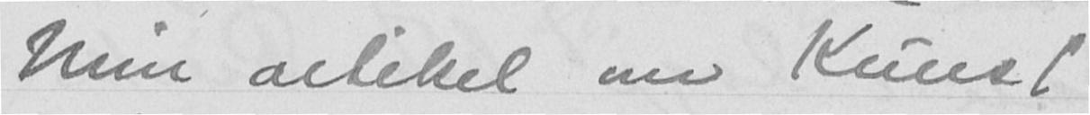Min artikel om Kunst
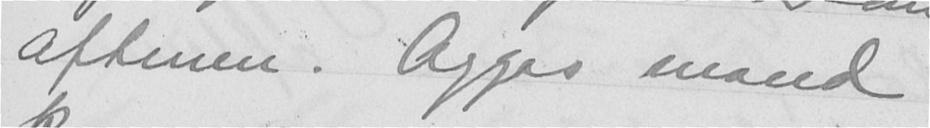aftenen. Aggas mand
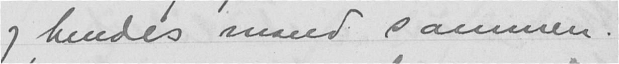og hendes mand sammen.
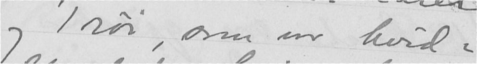og 1 røi, som var hvid-
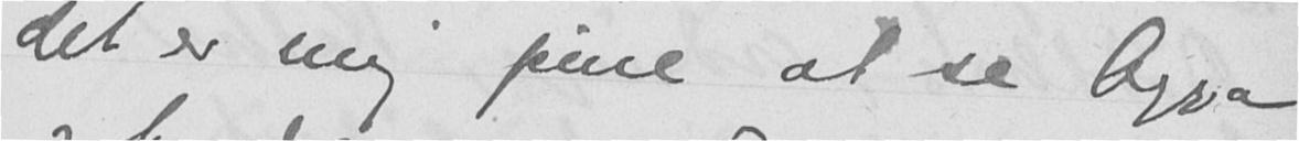det er mig pine at se Agga
